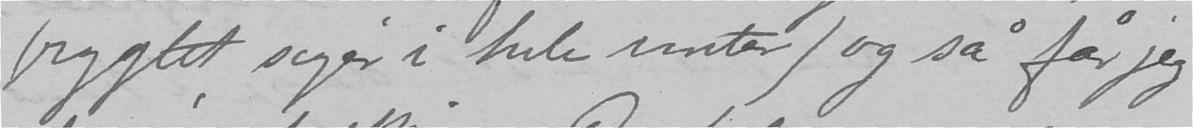(rygtet siger i hele vinter) og så får jeg
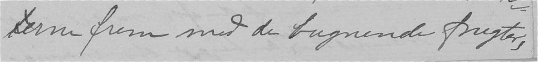terne frem med de bugnende frugter,
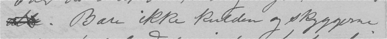ab. Bare ikke kulden og skyggerne
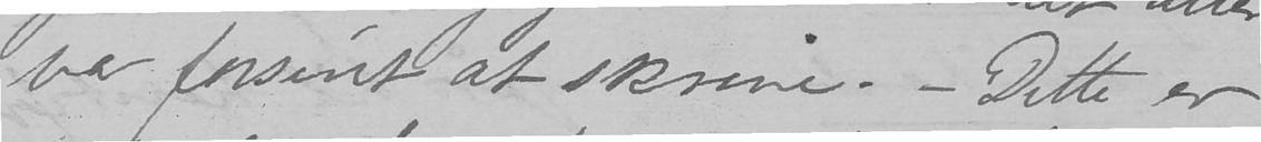var forsent at skrive. - Dette er
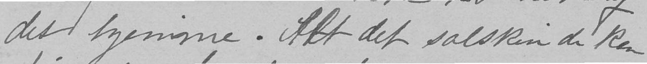det hjemme. Alt det solskin de kan
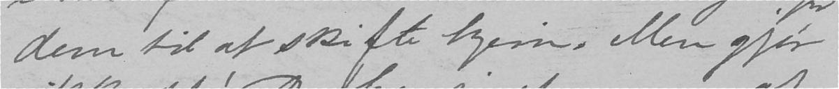dem til at skifte hjem. Men gjør
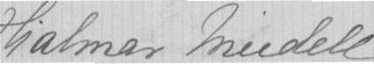Hjalmar Meidell
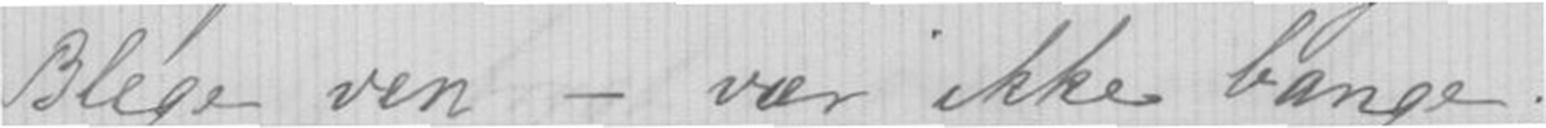Blege ven - vær ikke bange ,
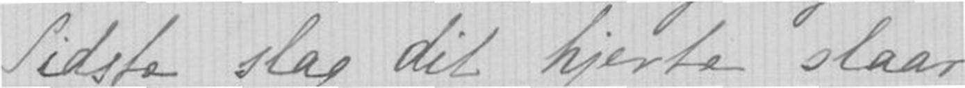Sidste slag dit hjerte slaar.
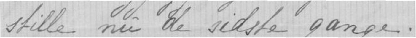stille nu de sidste gange.
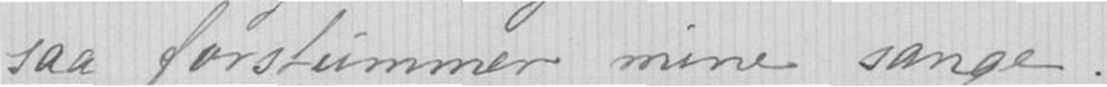saa forstummer mine sange.
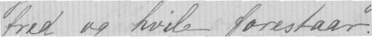fred og hvile forestaar.
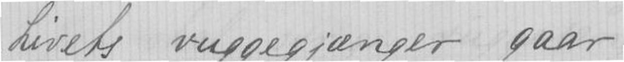Livets vuggegjænger gaar
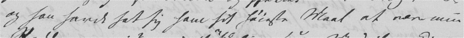og han havde sat sig som sit høieste Maal at være min
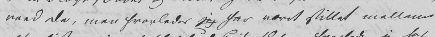veed De, men hvorledes jeg har været stillet mellem
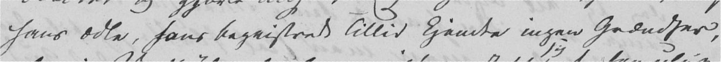hans ædle, hans begeistrede Tillid kjendte ingen Grændser,
